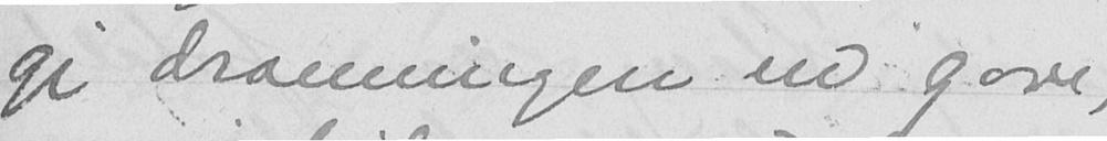gi dronningen en gave,
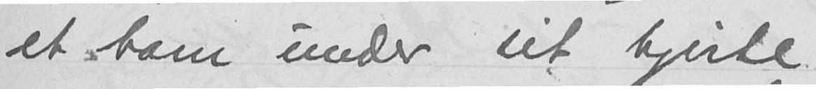et barn under sit hjerte
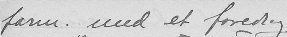form. med et foredrag
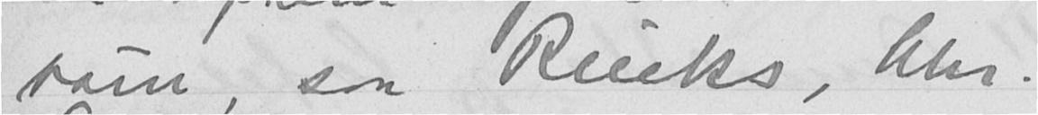børn, saa Riecks, Chr.
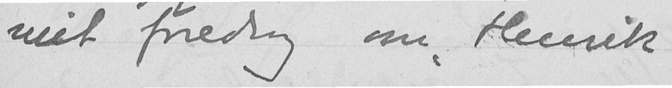mit foredrag om "Henrik
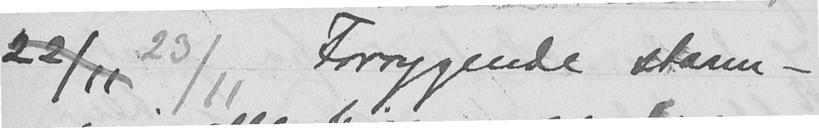22/11 23/11 Forrygende storm -
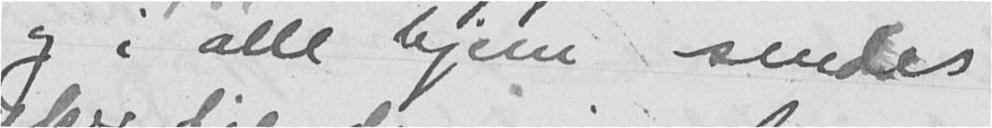og i alle hjem sendes
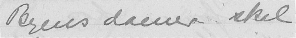Byens damer skal
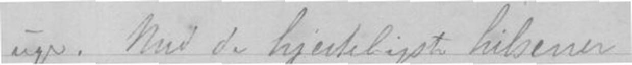Onsdag i næste uge. Med de hjerteligste hilsener
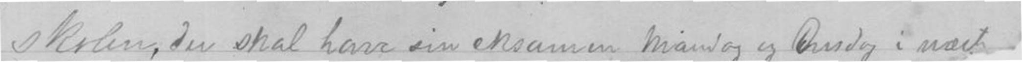skolen, der skal have sin eksamen Mandag og
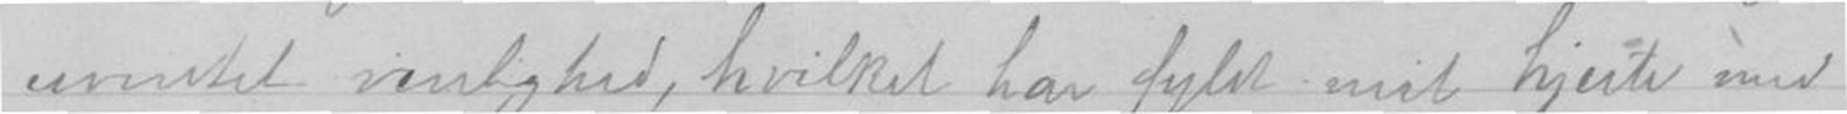uventet venlighed, hvilket har fyldt mit hjerte med
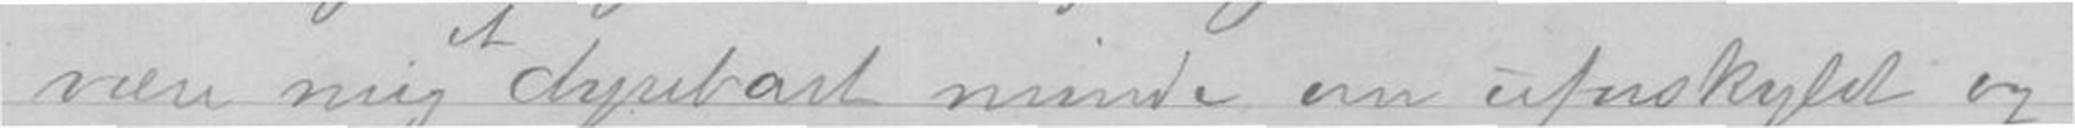være mig et dyrebart minde om uforskyldt og
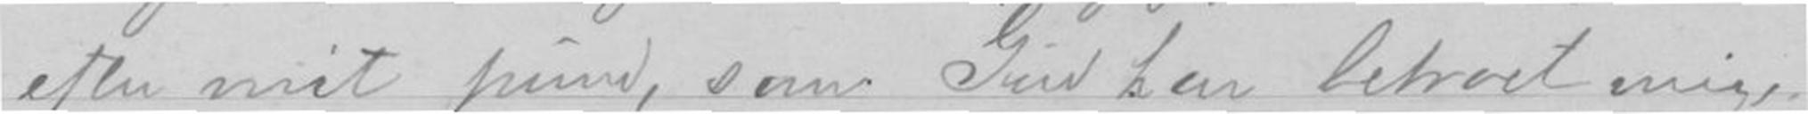efter mit pund, som Gud har betroet mig,
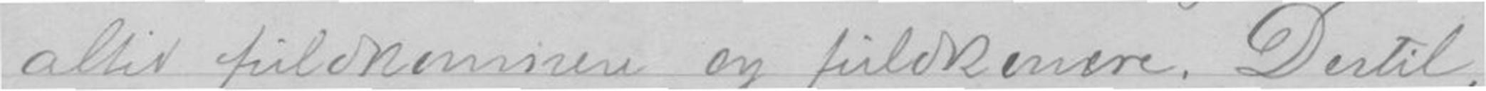altid fuldkommen og fuldkomere. Dertil,
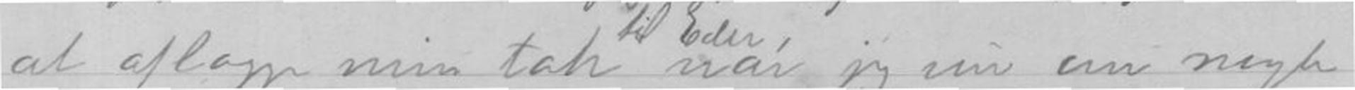at aflægge min tak når jeg nu om nogle
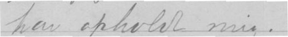har opholdt mig.
